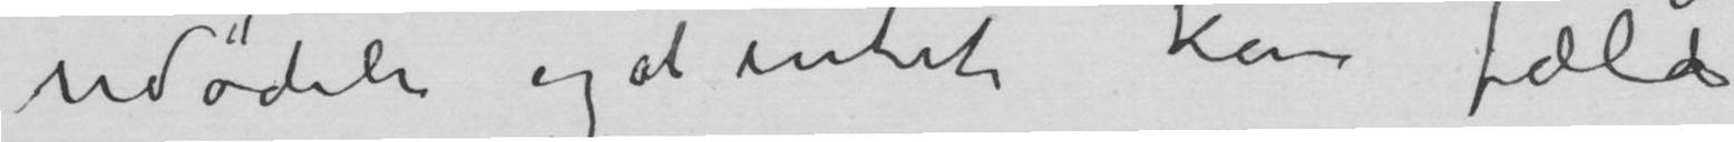udødeli og at intet kan fælde
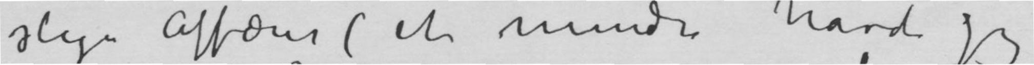slige Affærer (et mindre havde jeg
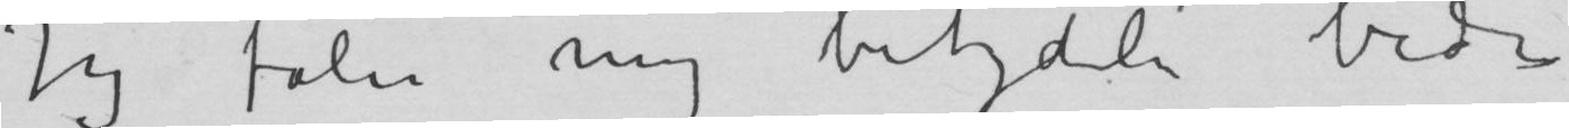Jeg føler mig betydli bedre
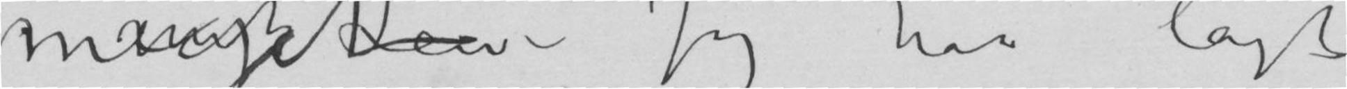meget – Jeg har lagt
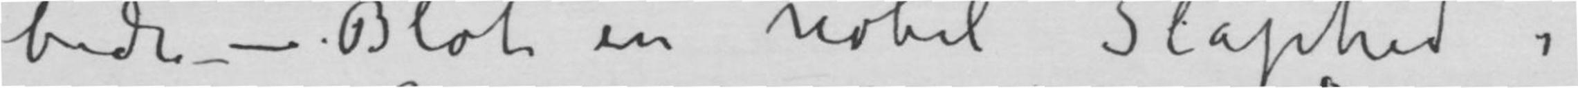bedre – Blot en nobel Slaphet i
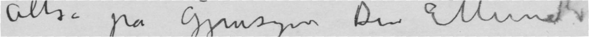Altså på gjensyn Din E Munch
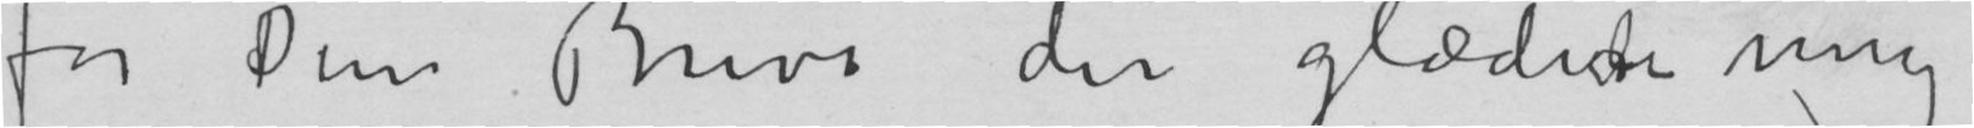for Dine Breve der glædede mig
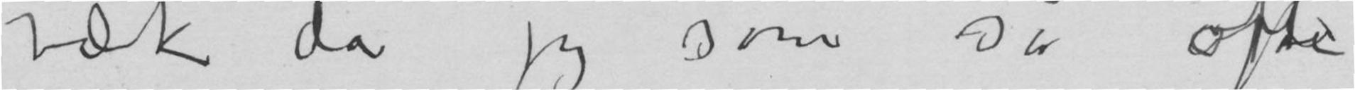væk da jeg som så ofte
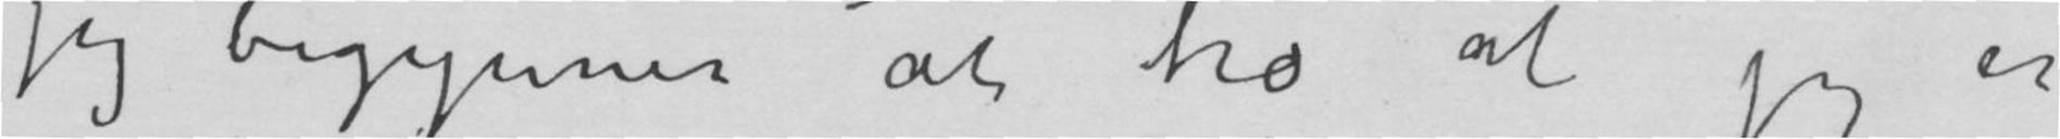jeg begynner at tro at jeg er
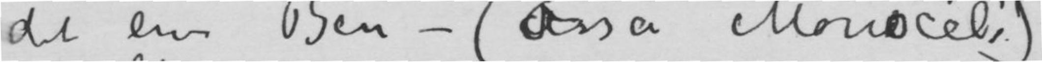det ene Ben – (osså Monocel!)
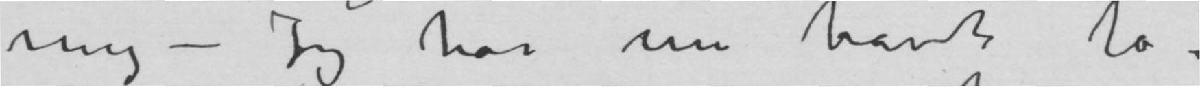mig – Jeg har nu havt to
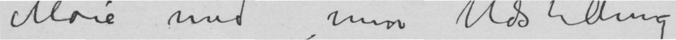Møie med min Udstilling –
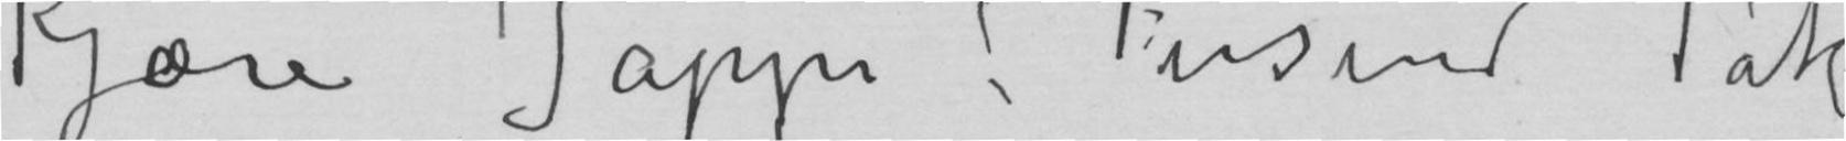Kjære Jappe! Tusind Tak
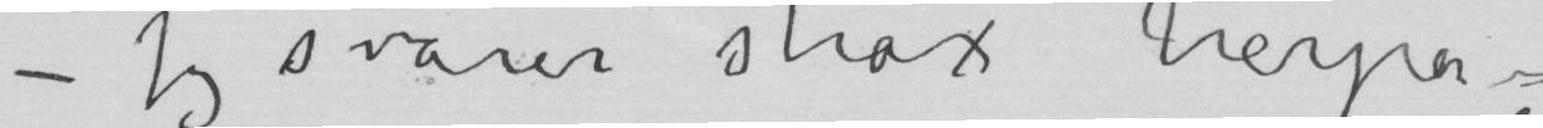– Jeg svarer strax herpå –
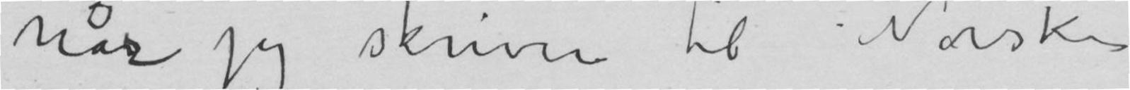når jeg skriver til Norske
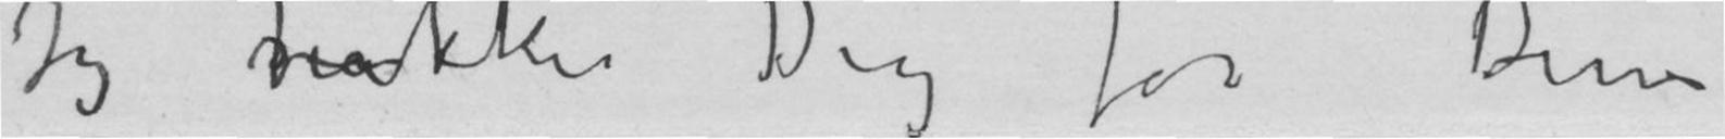Jeg takker Dig for Din
